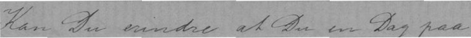Kan Du erindre, at Du en Dag paa
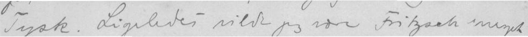Tysk. Ligeledes vilde jeg være Fritzsch meget
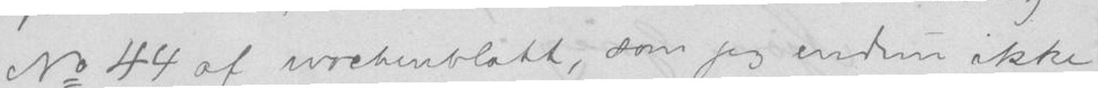N=44 af wochenblatt, som jeg endnu ikke
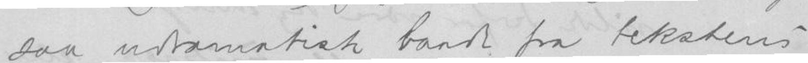saa udramatisk baade fra tekstens
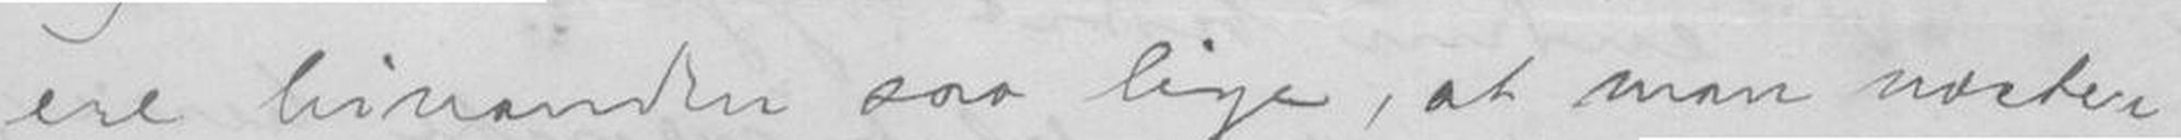ere hinanden saa lige, at man næsten
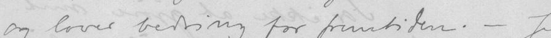og lover bedring for fremtiden.- Jeg
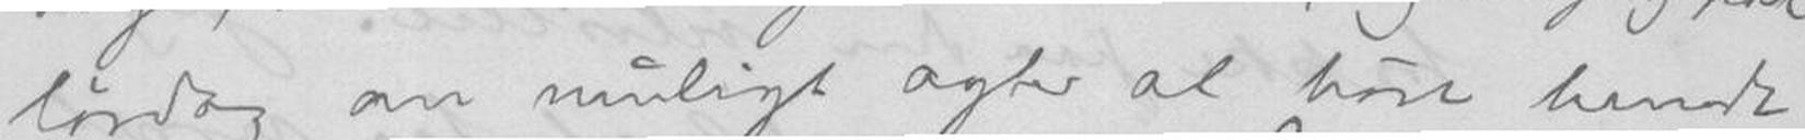lørdag om muligt agter at høre hende
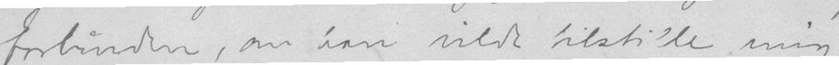forbunden, om han vilde tilstille mig
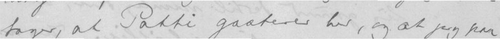tager, at Patti gaaterer her, og at jeg paa
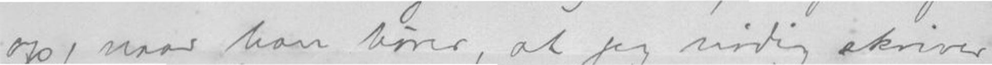op,naar han hører, at jeg nødig skriver
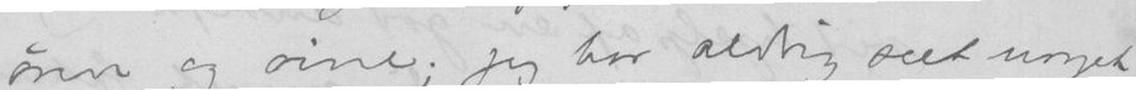øren og øine; jeg har aldrig seet noget
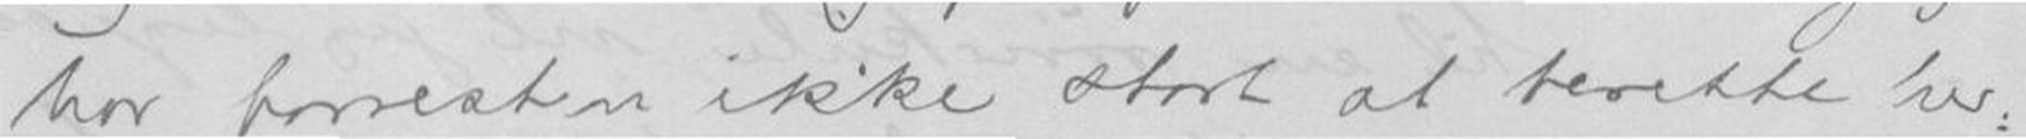har forresten ikke stort at berette her-
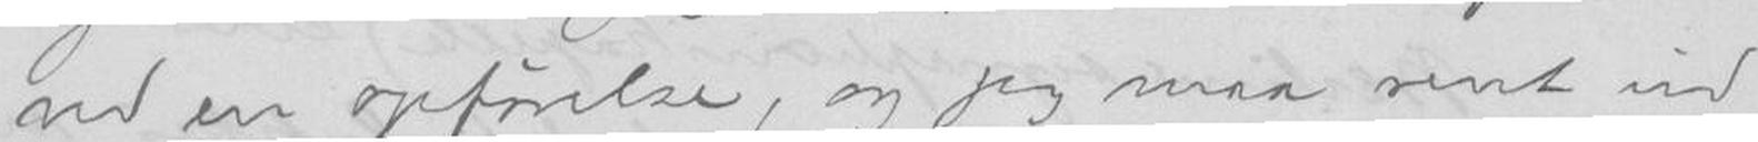med en opførelse, og jeg maa rent ud
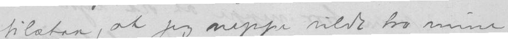tilstaa, at jeg neppe vilde tro mine
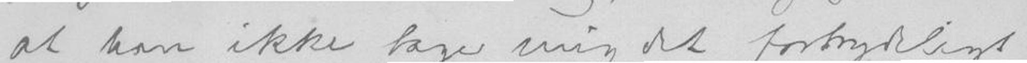at han ikke tager mig det fortrydeligt
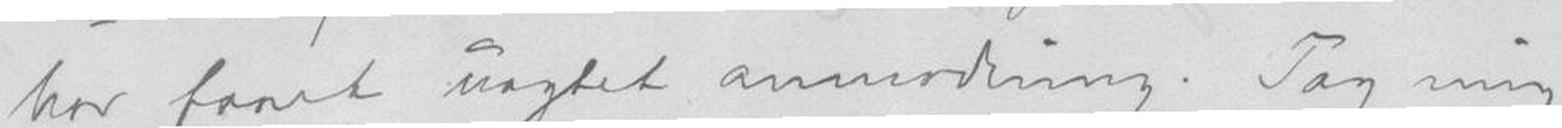har faaet uagtet anmodning. Tag mig
